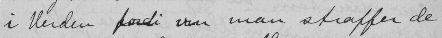i verden fordi  man straffer de
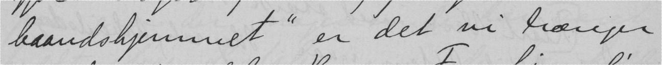baandshjemmet" er det vi trænger
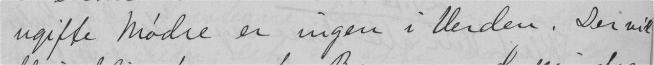ugifte mødre er ingen i verden. Der vil
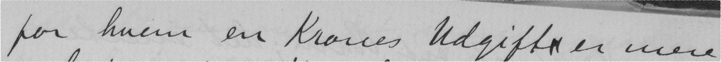for hvem en Krones udgift er mere
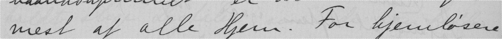mest af alle Hjem. For hjemløsere
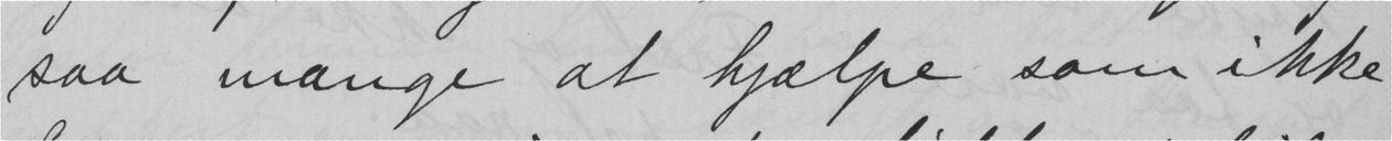saa mange at hjælpe som ikke
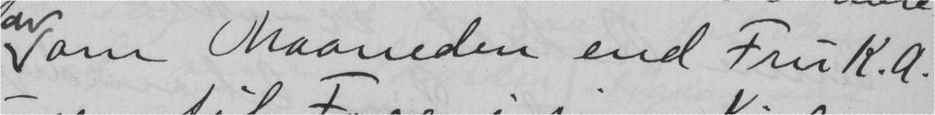om maaneden end Fru K. A.
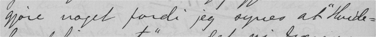gjøre noget fordi jeg synes at "Hvide-
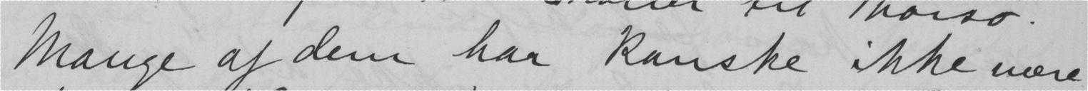Mange af dem har kanske ikke nære
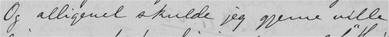Og alligevel skulde jeg gjerne ville
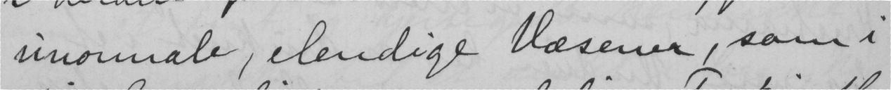unormale, elendige væsener, som i
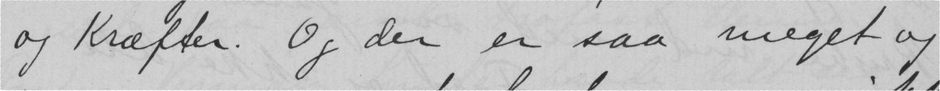og Kræfter. Og der er saa meget og
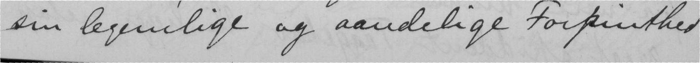sin legemlige og aandelige Forpinthed
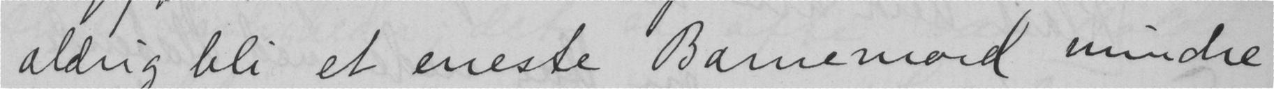aldrig bli et eneste Barnemord mindre
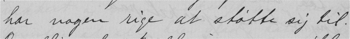har nogen rige at støtte sig til.
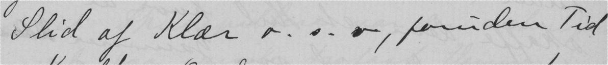Slid af Klær o. s. v, foruden Tid
